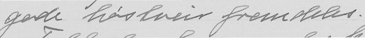gode høstveir fremdeles.
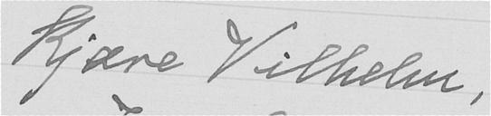Kjære Vilhelm,
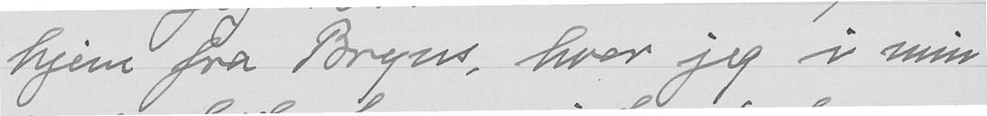hjem fra Bryns, hvor jeg i min
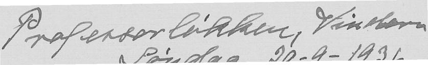Professerløkken, Vindern
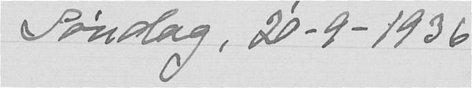Søndag, 20-9-1936
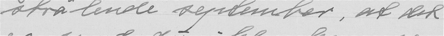strålende september, at det
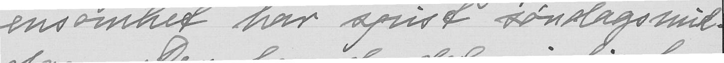ensomhet har spist søndagsmid-
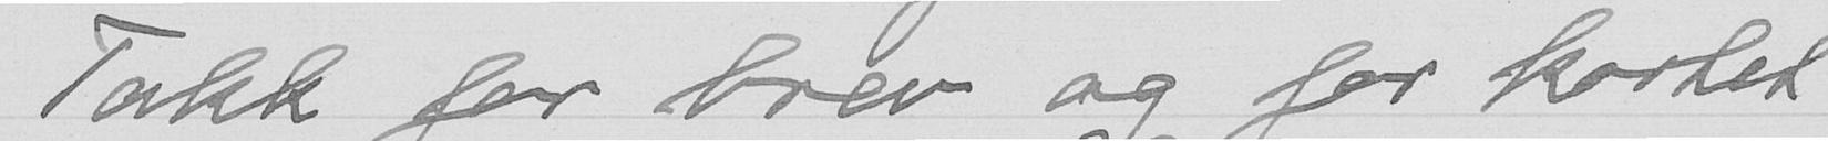Takk for brev og for kortet
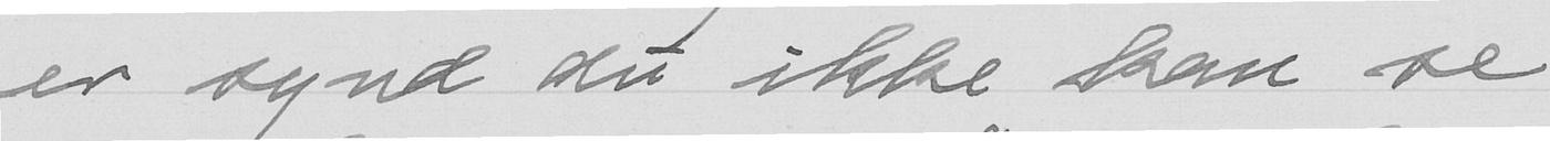er synd du ikke kan se
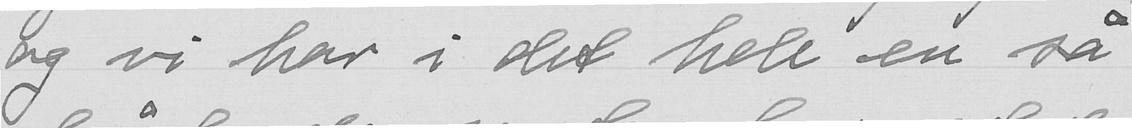- og vi har i det hele en så
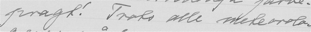pragt! Trots alle meteorolo-
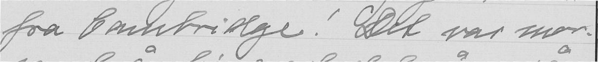fra Cambridge. Det var mor-
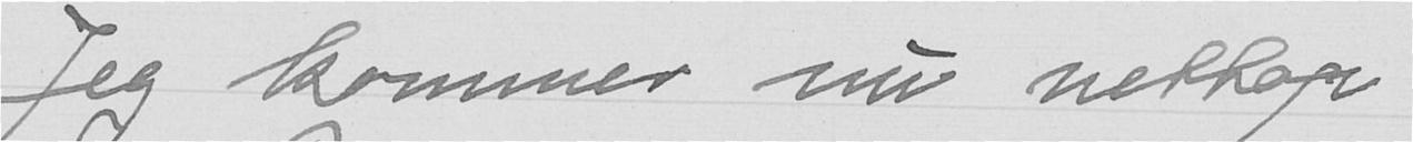Jeg kommer nu nettop
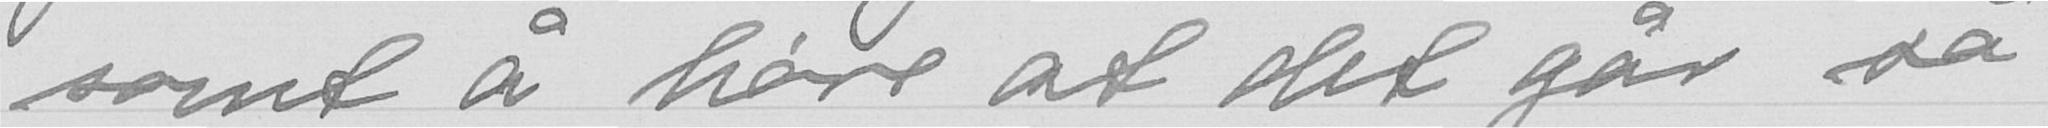somt å høre at det gar så
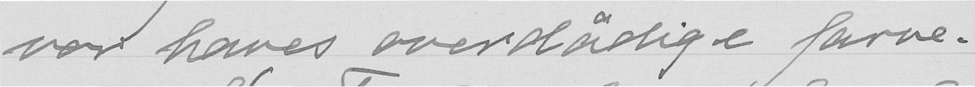vor haves overdådige farve-
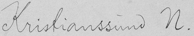Kristianssund N.
